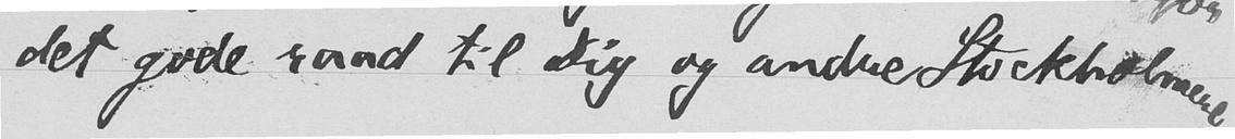det gode raad til Dig og andre Stockholmere,
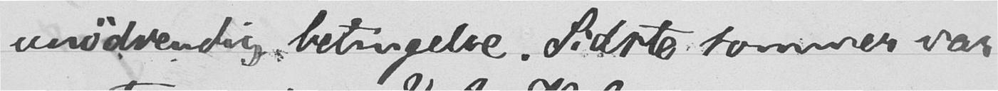unødvendig betingelse. Sidste sommer var
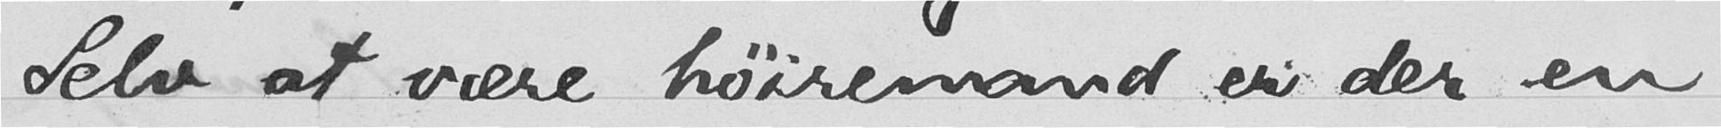Selv at være høiremand er der en
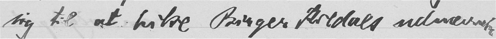sig til at hilse Birger Kildals udnævnelse
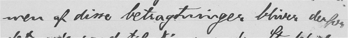men af disse betragtninger bliver derfor
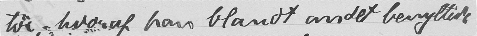tør, hvoraf han blandt andet benyttede
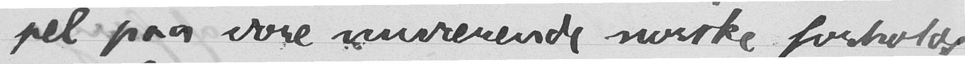pel paa vore nuværende norske forholds
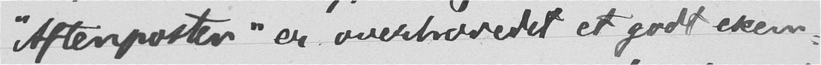"Aftenposten" er overhovedet et godt exem-
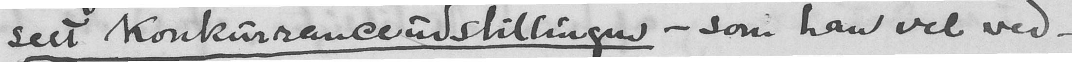seet konkurranceudstillingen - som han vel ved
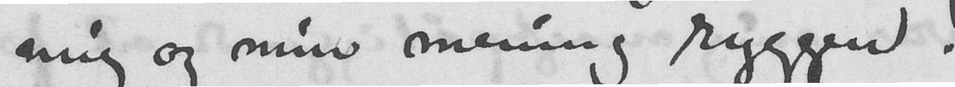mig og min mening ryggen!
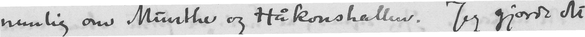nemlig om Munthe og Håkonshallen. Jeg gjorde det
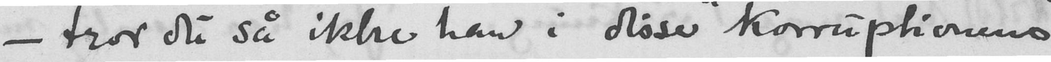- tror du så ikke han i disse "korruptionens"
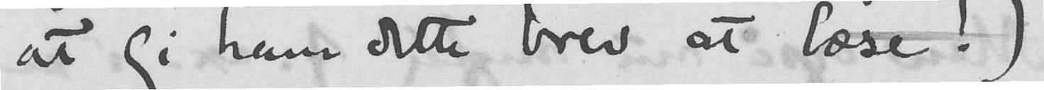at gi ham dette brev at læse!)
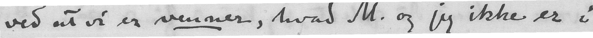ved at vi er venner, hvad M. og jeg ikke er i
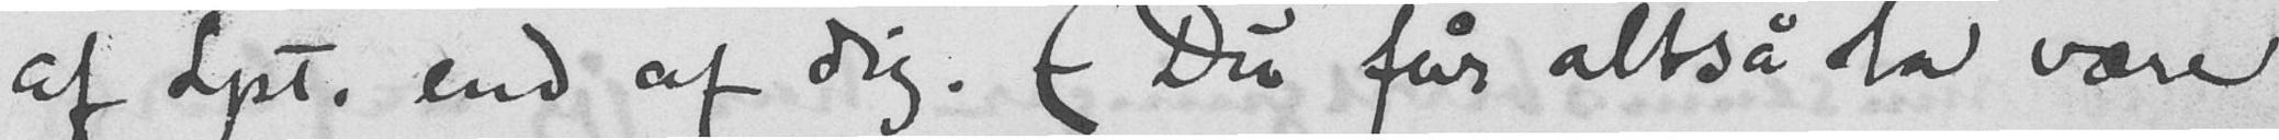af Lpt. end af dig. (Du får altså la være
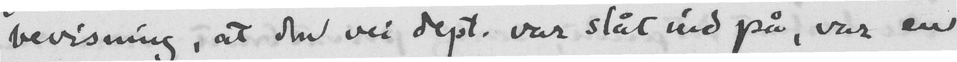bevisning, at den vei dept. var slåt ind på, var en
